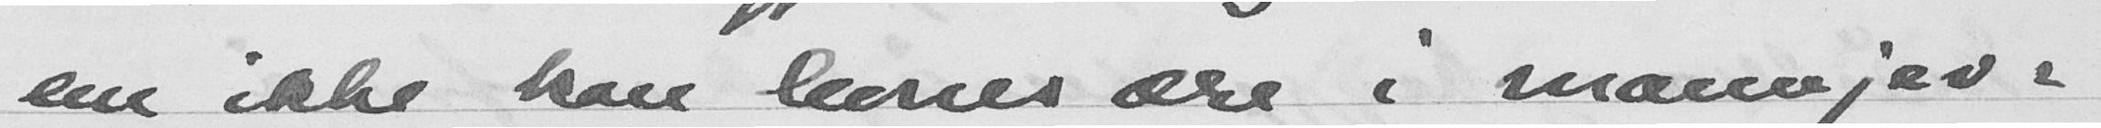ene ikke kan livnes ære i mamjas-
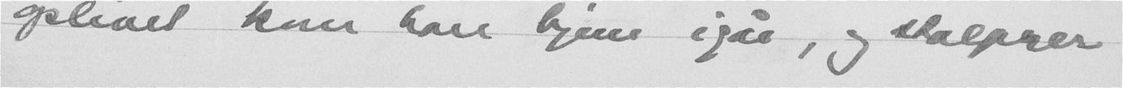oplivet kom han hjem igår, og stolprer
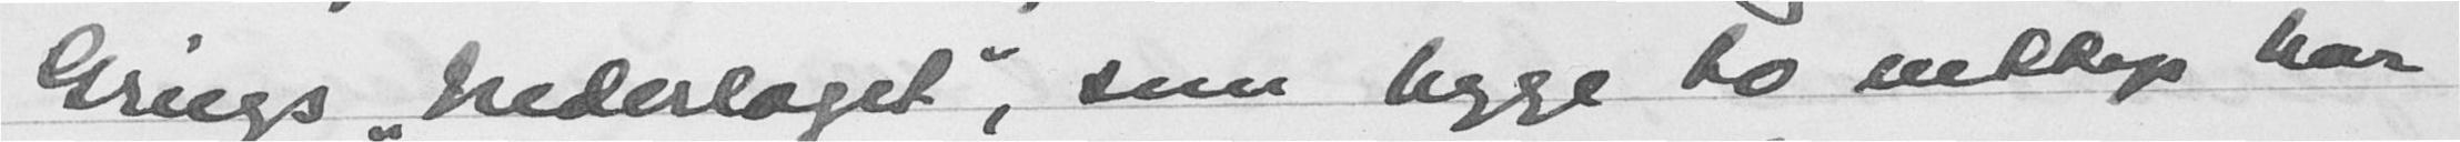Griegs "Nederlaget", som begge to nettop har
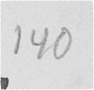140
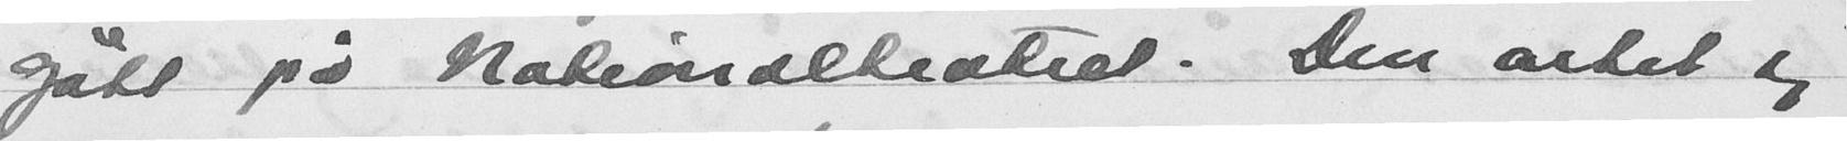gått på Nationalteatret. Den artet sig
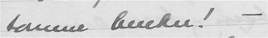tomme benker! -
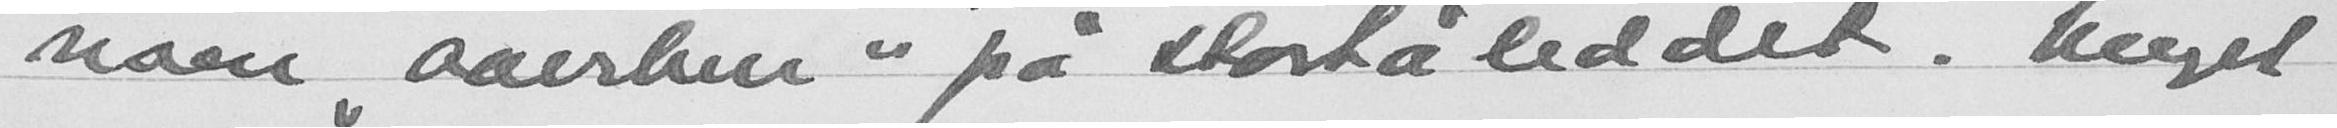noen "aaerbur" pa stortåleddet. Meget
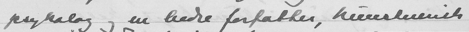psykolog og en bedre forfatter, kunstnerisk
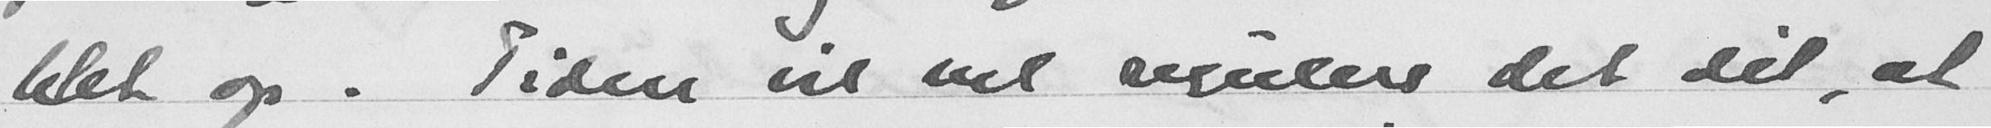let op. Tiden vil vel regulere det dit, at
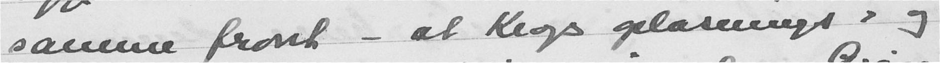samme front - at Krogs opløsnings og
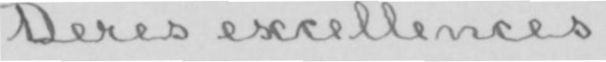Deres excellences
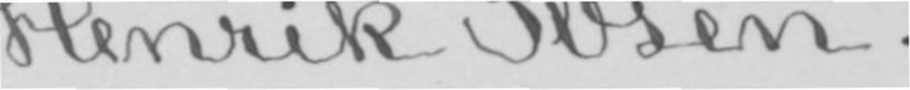Henrik Ibsen.
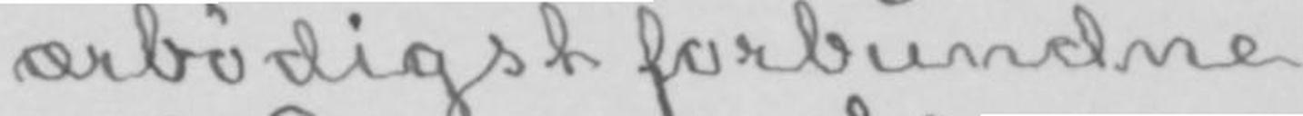ærbødigst forbundne
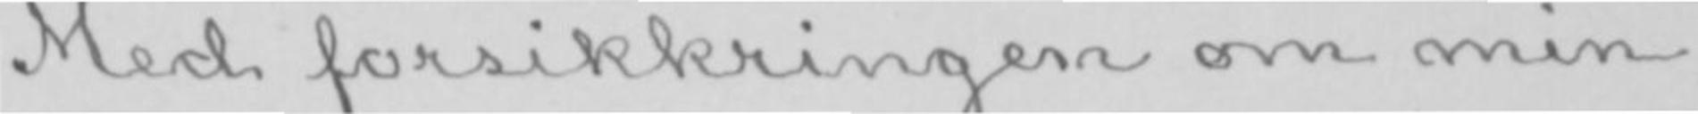Med forsikkringen om min
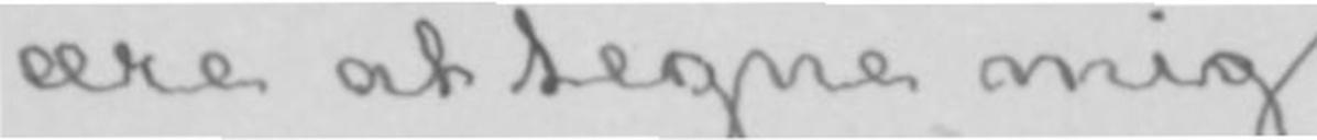ære at tegne mig
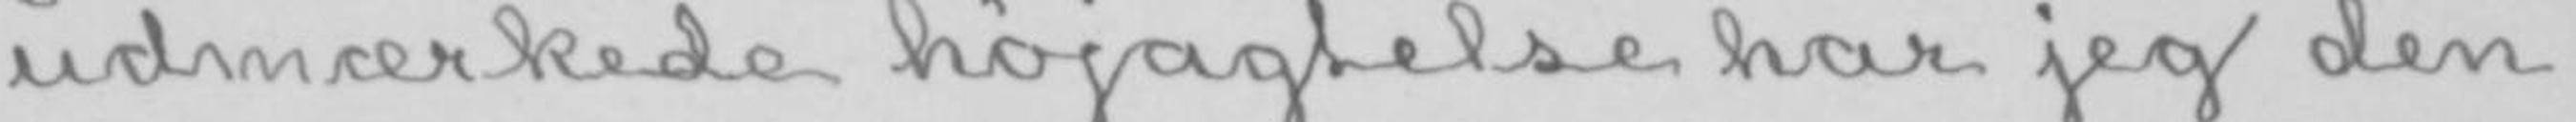udmærkede højagtelse har jeg den
